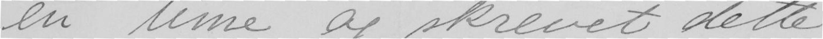en time og skrevet dette.
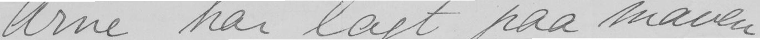Arne har lagt paa maven
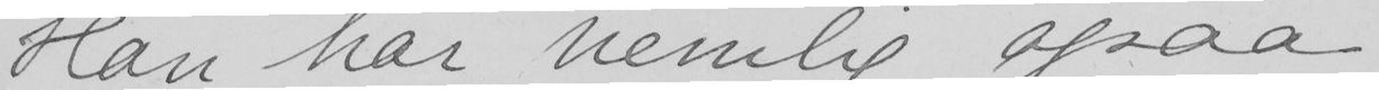Han har nemlig ogsaa
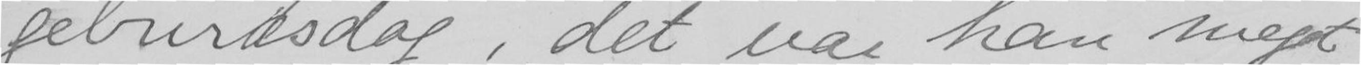geburdsdag , det var han meget
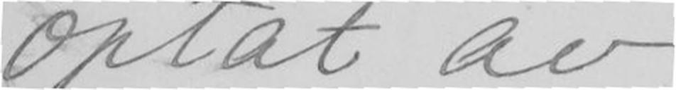optat av.
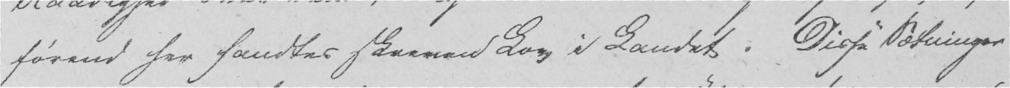førend har fandtes skreven Lov i Landet. Disse Sætninger
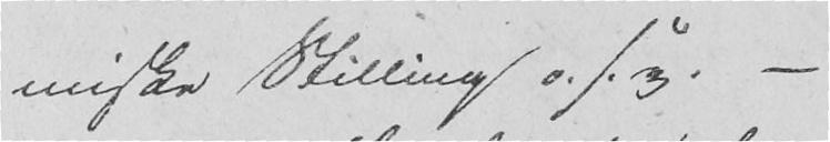miske Stilling o. s. v. -
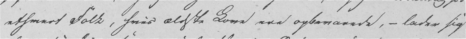ethvert Folk, hvis ældste Love var opbevarede, – lader sig
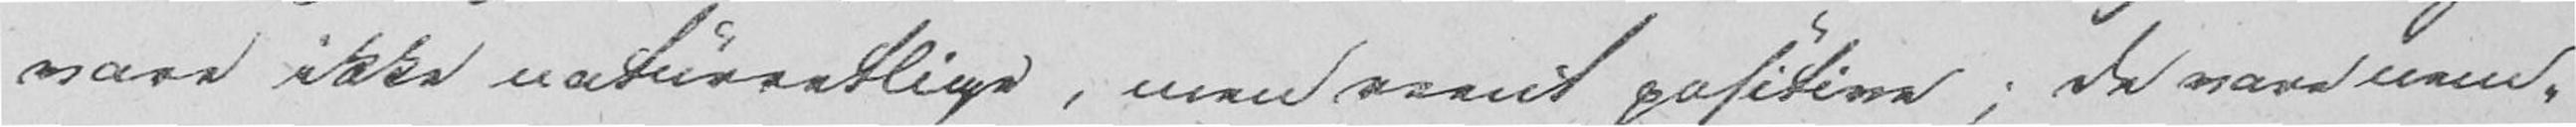vare ikke naturretlige, men reent positive; de vare nem-
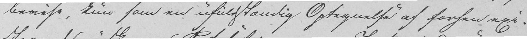bevise, kun som en ufuldstændig Optegnelse af forhen exi-
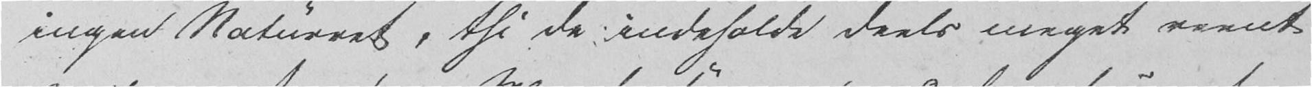ingen Naturret, thi de indeholde deels meget reent
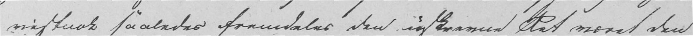vistnok saaledes fremdeles den uskrevne Ret været den
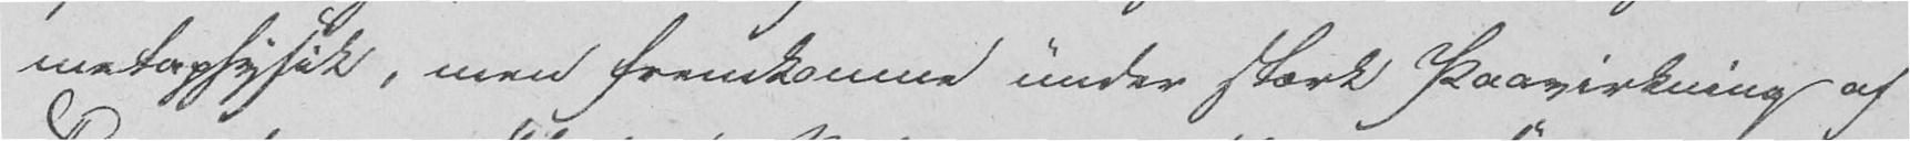metaphysik, men fremkomne under stærk Paavirkning af
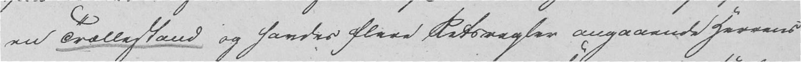en Trællestand og havdes flere Retsregler angaaende Herrens
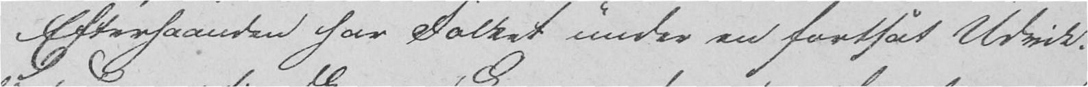Efterhaanden har Folket under en fortsat Udvidk-
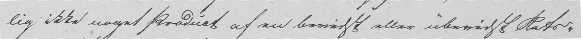lig ikke noget Product af en bevidst eller ubevidst Rets-
